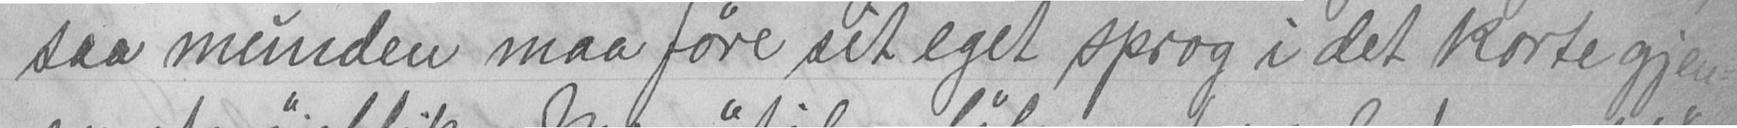saa munden maa føre sit eget sprog i det korte gjen-
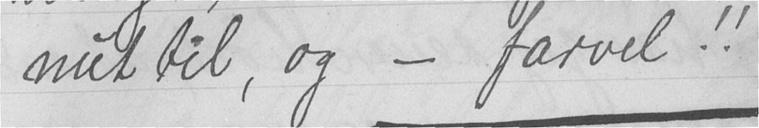nut til, og - farvel !!
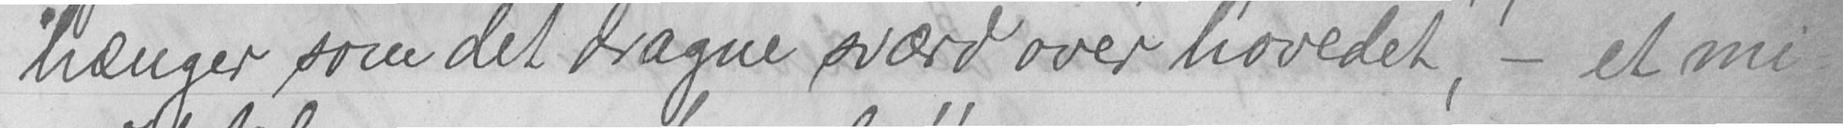hænger som det dragne sværd over hovedet, - et mi-
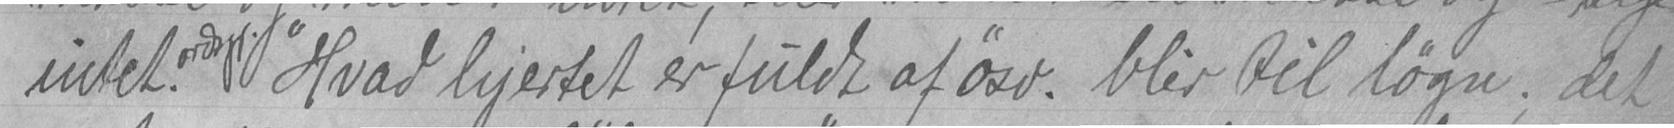intet. ord "Hvad hjertet er fuldt af" osv. blir til løgn; det
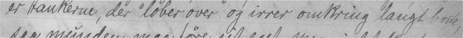er tankerne, der "løber over" og irrer omkring langt borte,
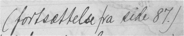(fortsættelse fra side 87.)
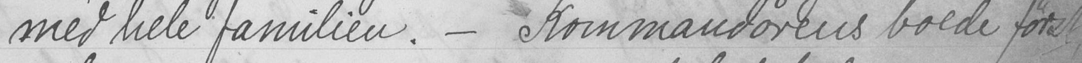med hele familien. - Kommandørens boede først,
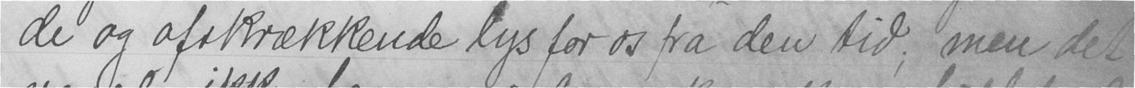de og afskrækkende lys for os fra den tid; men det
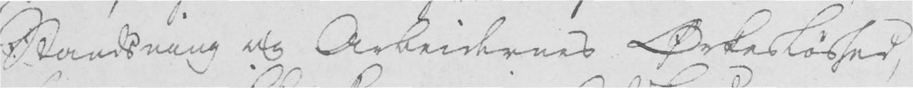Standsning og Arbeidernes Ørkesløshed,
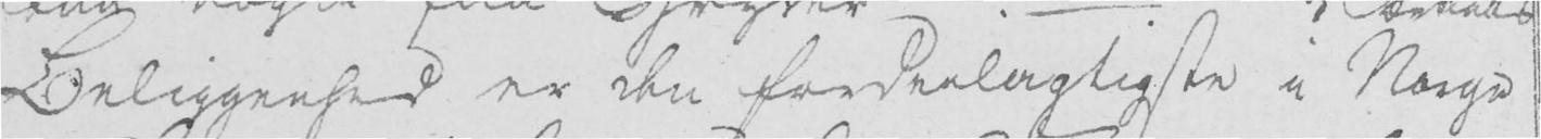Beliggenhed er den fordeelagtigste i Norge
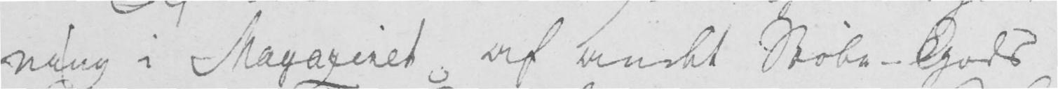ning i Magazinet; af andet Støbe-Gods
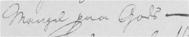Mangel paa Gods -
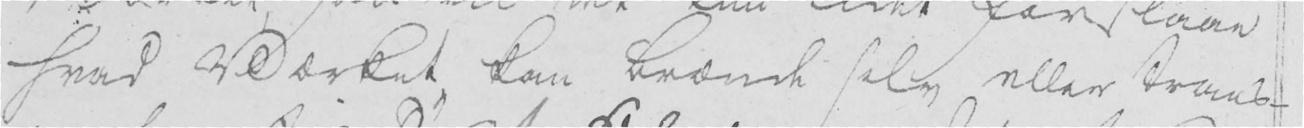hvad Værket kan brænde selv eller trans-
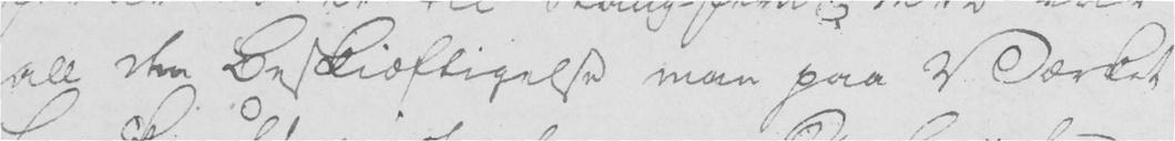all den Beskiæftigelse man paa Værket
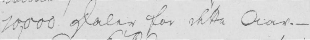20,000 Daler for dette Aar. -
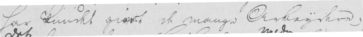har kundet givet de mange Arbeydere;
